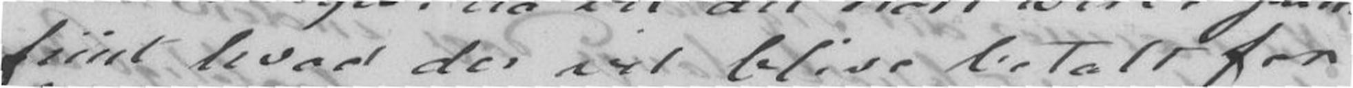hvad det vil blive betalt for
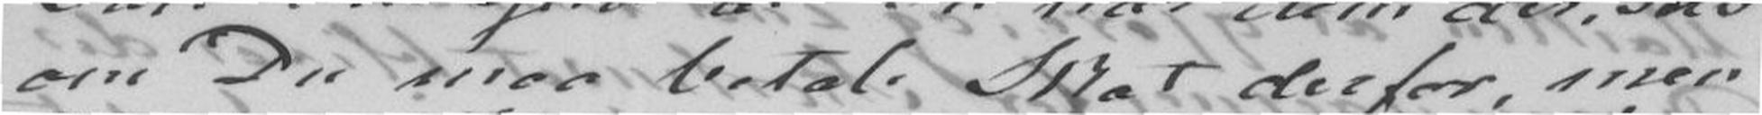om Du maa betale Skat derfor, men
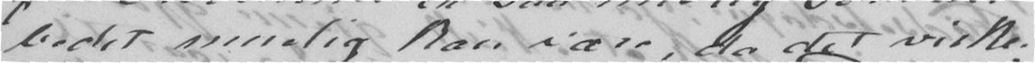bedst muelig kan være, da det virke-
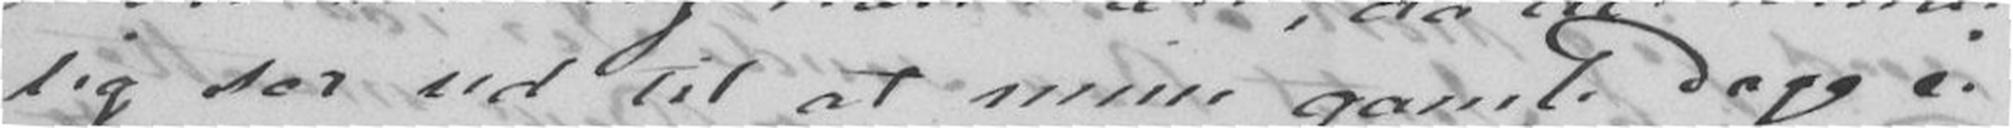lig ser ud til at mine gamle Dage ei
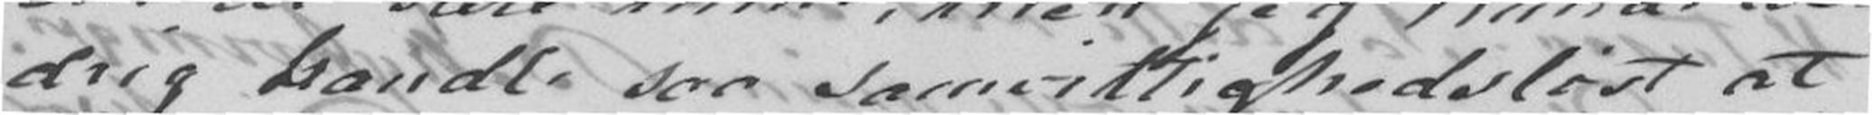drig handle saa samvittigsløst at
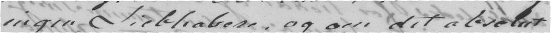ingen Liebhabere, og om det absolut
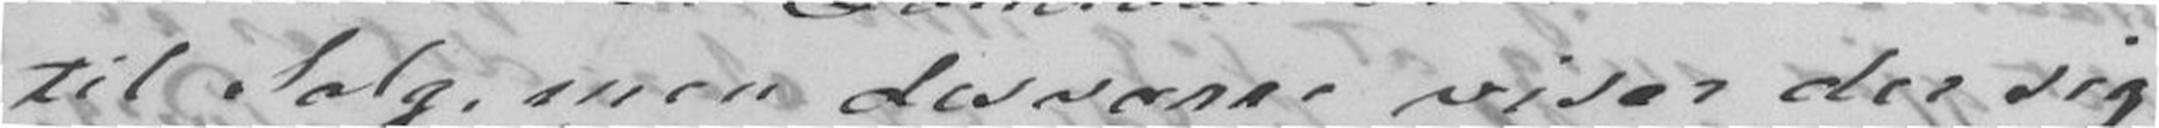til Salg, men desværre viser der sig
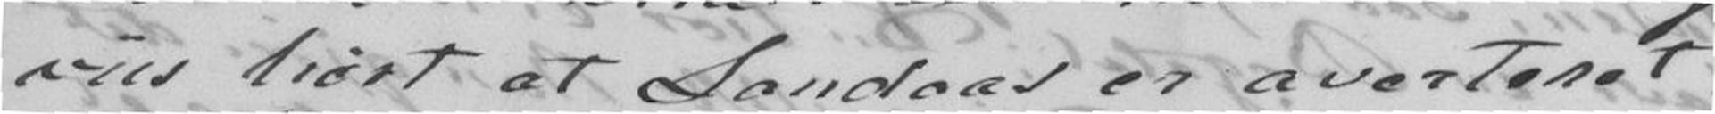viis hørt at Landaas er averteret
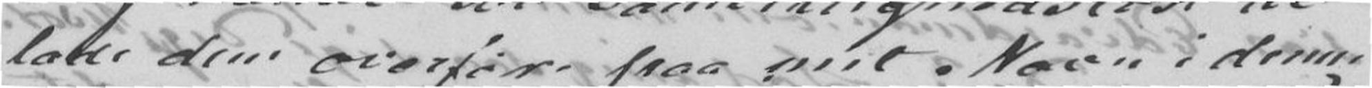lade dem overføres paa mit Navn i denne
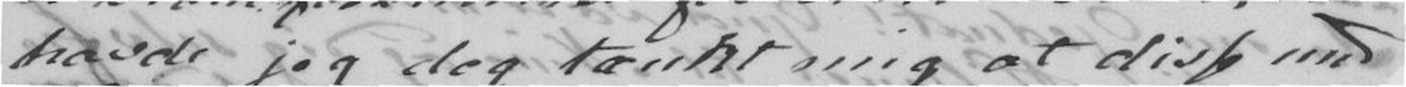havde jeg dog tænkt mig at disse med
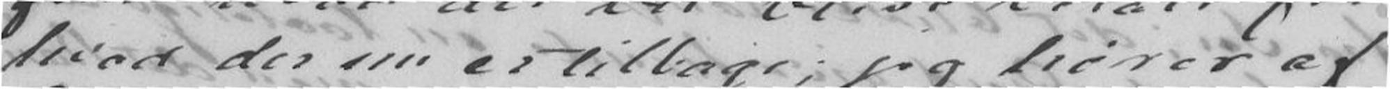fiint hvad der nu er tillage; jeg hører af
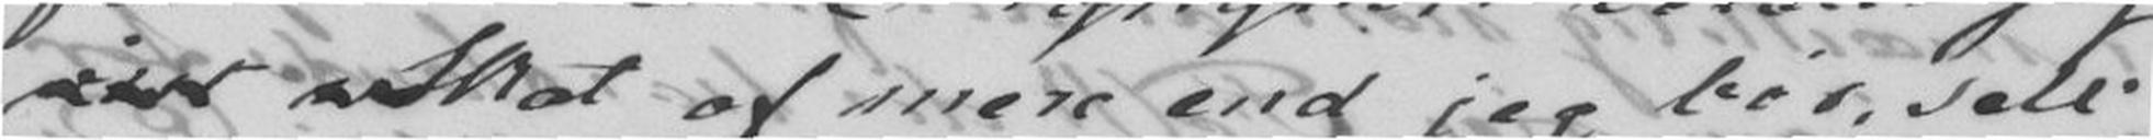vist Skat af mere end jeg bør, selv
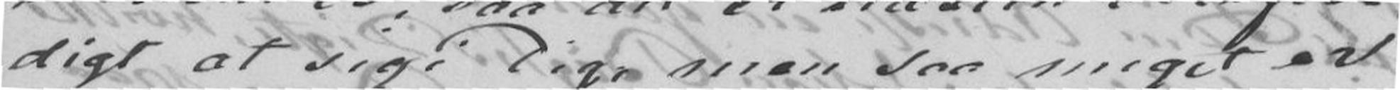digt at sige Dig, men saa meget er
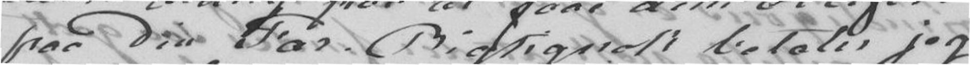paa Din Far. Rigtignok betaler jeg
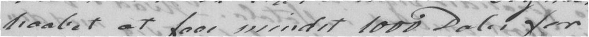haabet at faae mindst 1000 Daler for
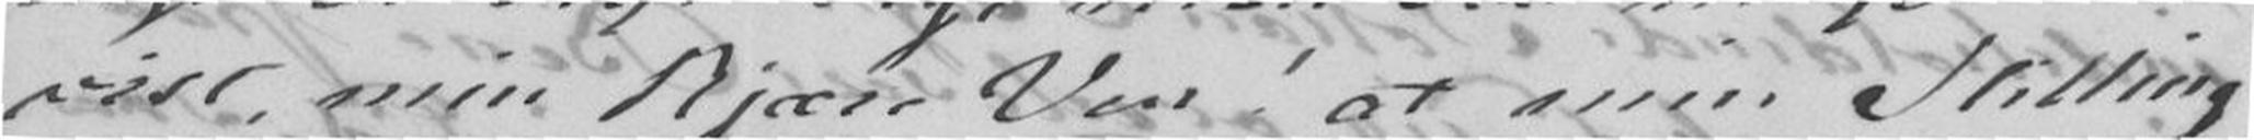vist, min kjære Ven! at min Stilling
